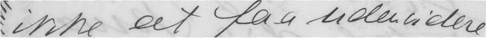ikke at faa udenvidere
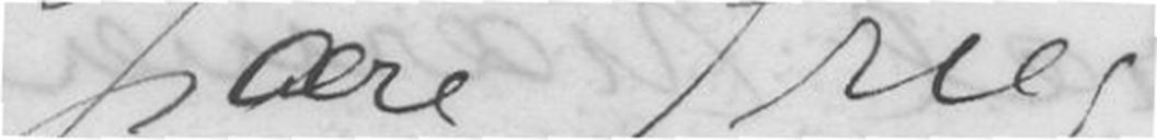Kjære Grieg.
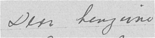Din hengivne
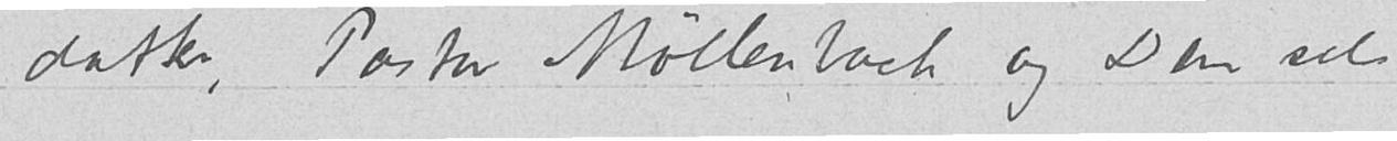datter, Pastor Møllenbeck og Dem selv
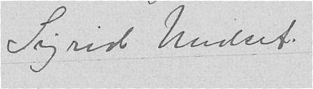Sigrid Undset.
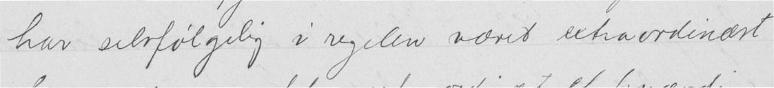har selvfølgelig i regelen været extraordinært
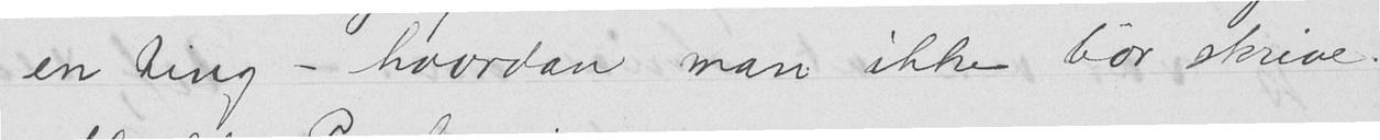en ting - hvordan man ikke bør skrive.
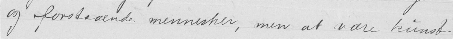og forstaaende mennesker, men at være kunst-
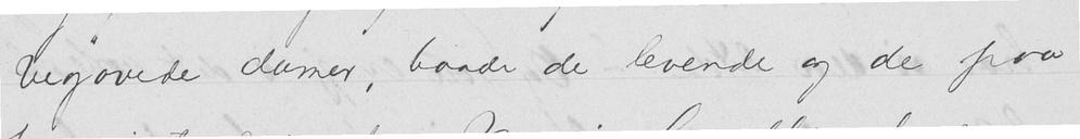begavede damer, baade de levende og de paa
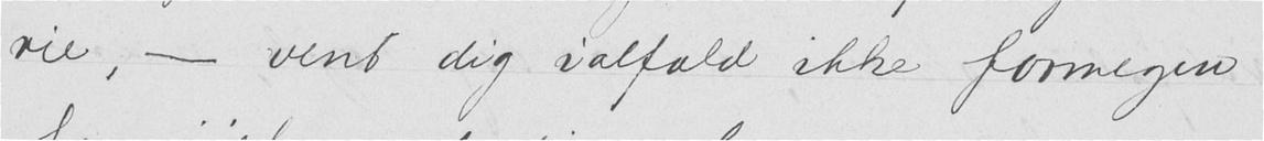rie, - vent dig ialfald ikke formegen
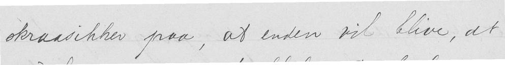skraasikker paa, at enden vil blive, at
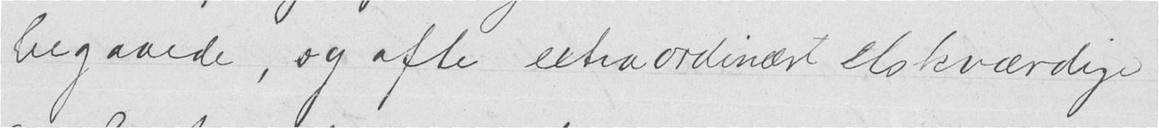begavede, og ofte extraordinært elskværdige
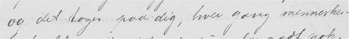- og det tager paa dig, hver gang mennesker-
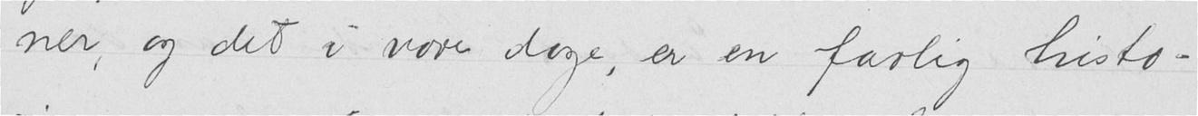ner, og det i vore dage, er en farlig histo-
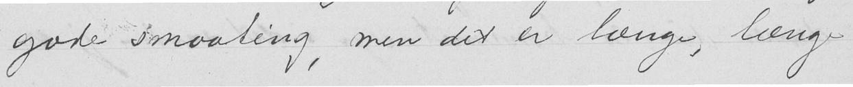gode smaating, men det er længe, længe
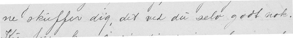ne skuffer dig, det ved du selv godt nok.
